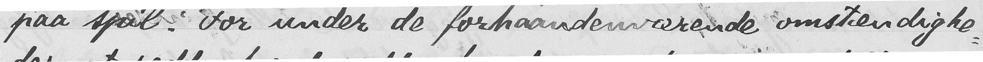paa spil. For under de forhaandenværende omstændighe-
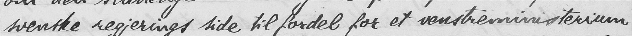svenske regerings side til fordel for et venstreministerium
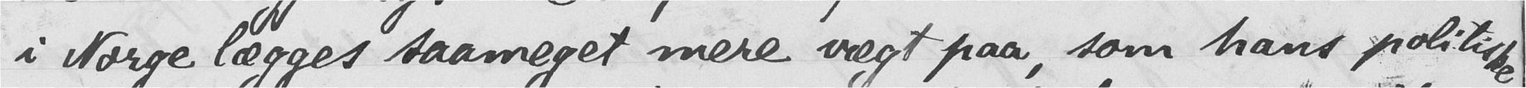i Norge lægges saameget mere vægt paa, som hans politiske
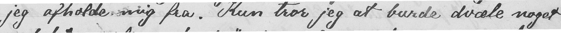jeg afholde mig fra. Kun tror jeg at burde dvæle noget
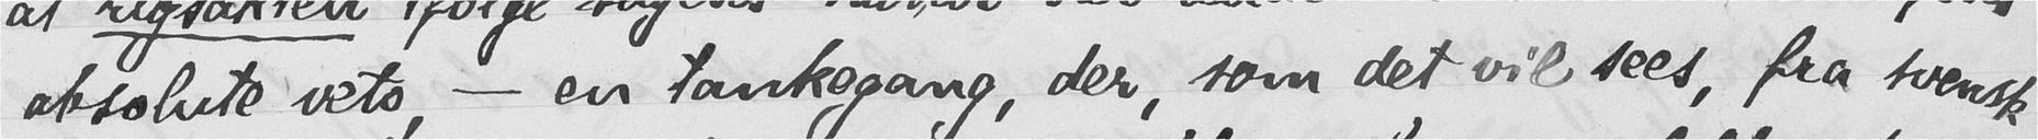absolute veto, - en tankegang, der, som det vil sees, fra svensk
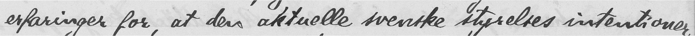erfaringer for, at den aktuelle svenske styrelses intentioner,
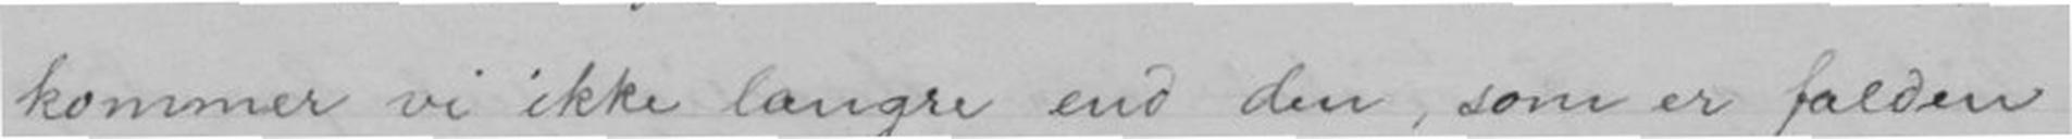kommer vi ikke længre end den, som er falden
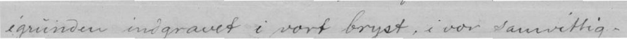igrunden indgravet i vort bryst, i vor samvittig-
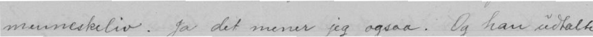menneskeliv. Ja det mener jeg ogsaa. Og han udtalte
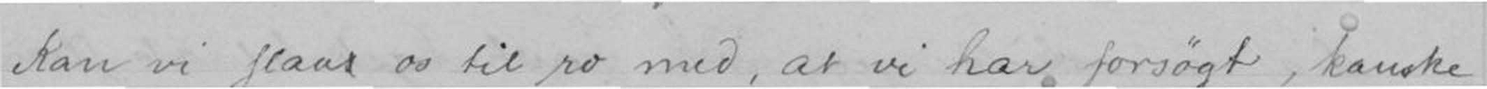Kan vi slaa os til ro med, at vi har forsøgt, kanske
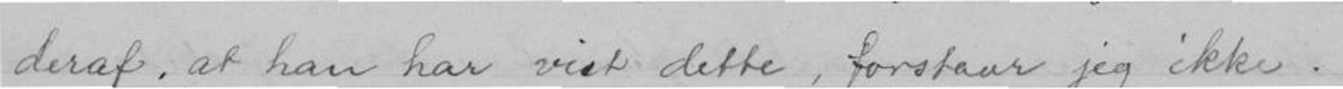deraf, at han har vist dette, forstaar jeg ikke.
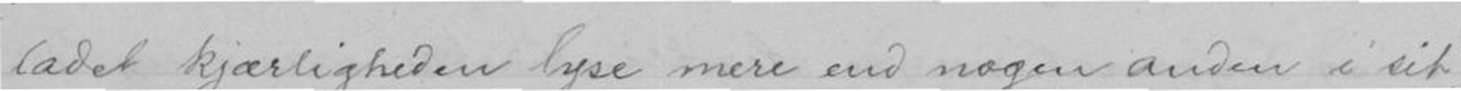ladet kjærligheden lyse mere end nogen anden i sit
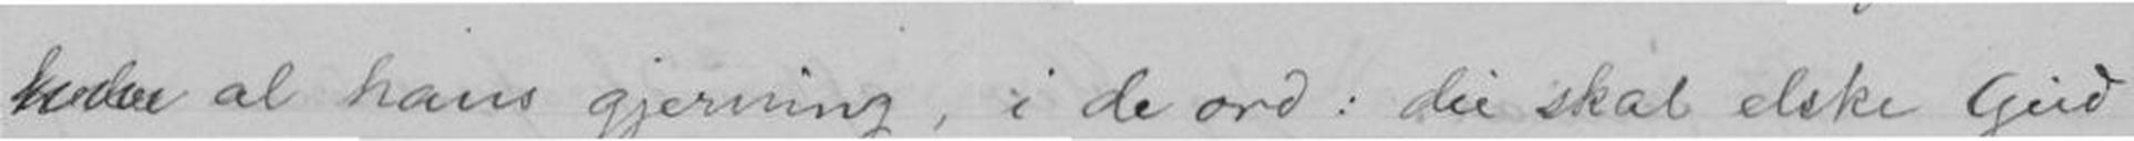al hans gjerning, i de ord: du skal elske Gud
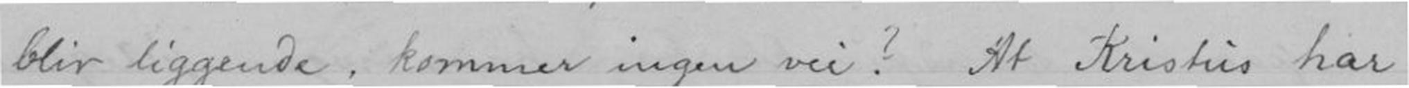blir liggende, kommer ingen vei? At Kristus har
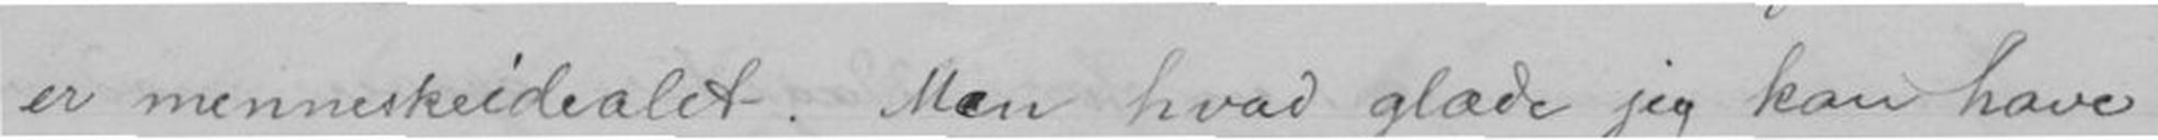er menneskeidealet. Men hvad glæde jeg kan have
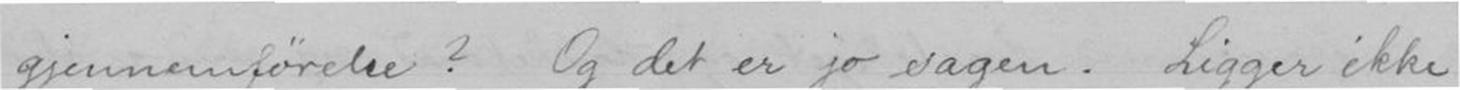gjennemførelse? Og det er jo sagen. Ligger ikke
